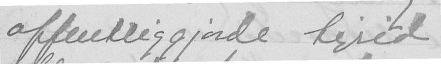offentliggjorde Sigrid
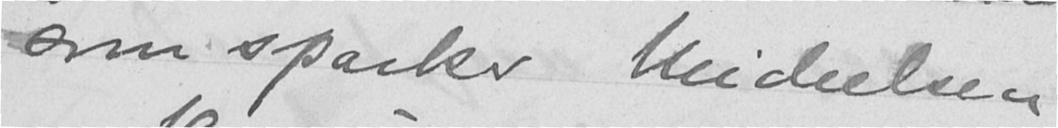som sparker Michelsen
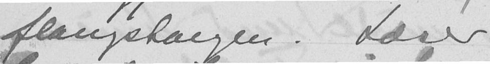flangstangen. Læser
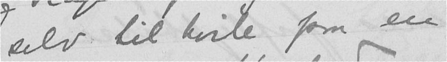selv til hvile paa en
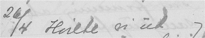26/4 Hvilte vi ud og
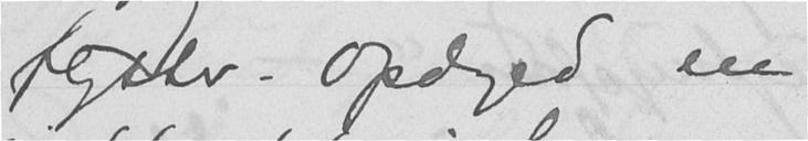flytter. Opdaged en
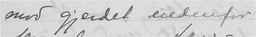mod gjerdet nedenfor
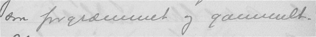saa forgræmmet og gammelt.
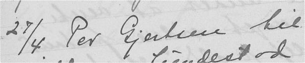27/4 Per Gjertsen til
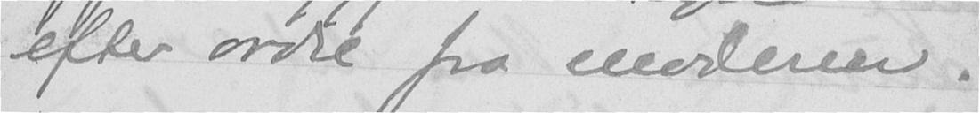efter ordre fra moderen.
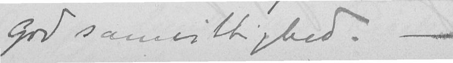god samvittighed. -
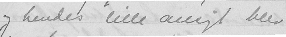og hendes lille ansigt blev
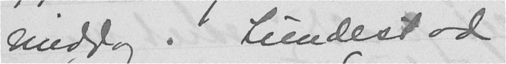middag. Lundestad
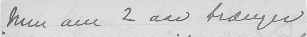"Men om 2 aar trænger
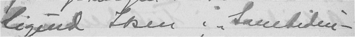Sigurd Ibsen i "Samtiden" -
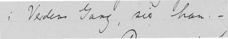i Verdens Gang, sier han. -
